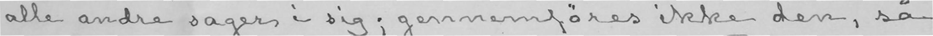alle andre sager i sig; gennemføres ikke den, så
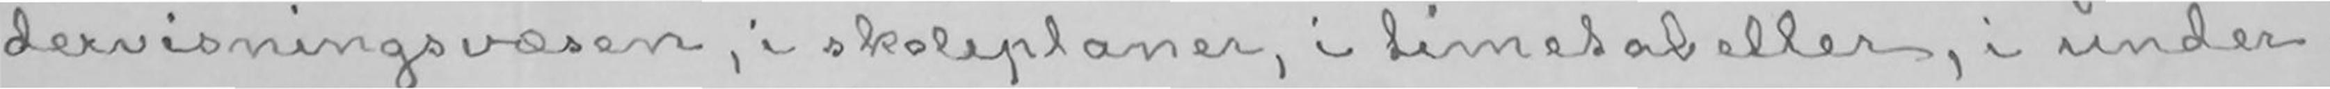dervisningsvæsen, i skoleplaner, i timetabeller, i under-
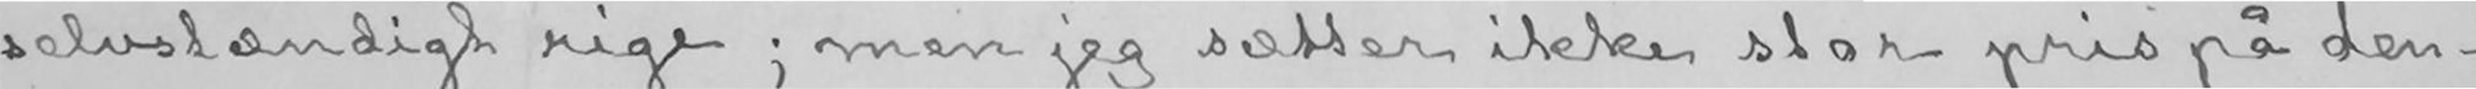selvstændigt rige; men jeg sætter ikke stor pris på den-
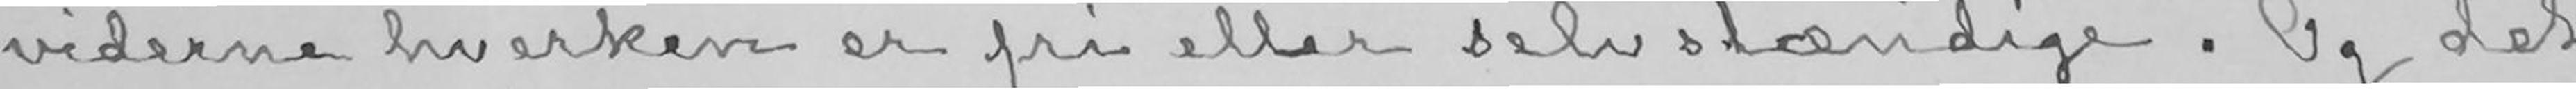viderne hverken er fri eller selvstændige. Og det
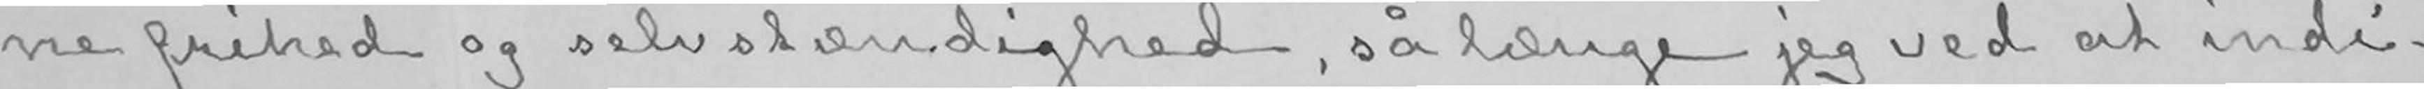ne frihed og selvstændighed, sålænge jeg ved at indi-
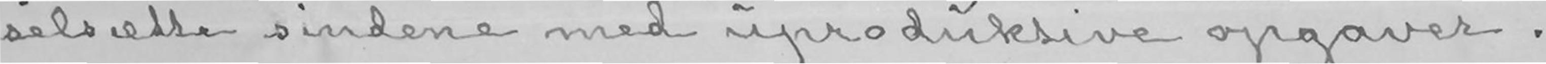selsætte sindene med uproduktive opgaver.
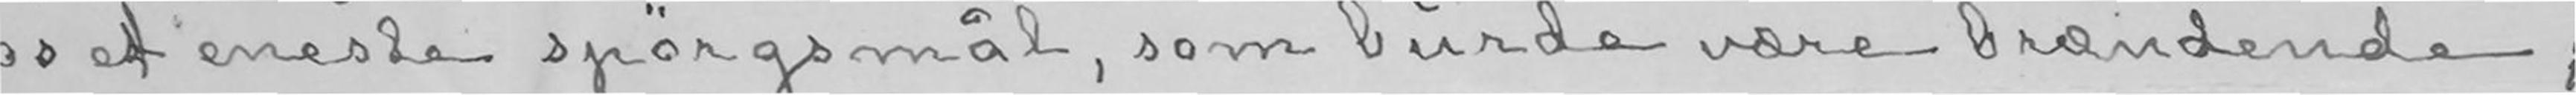os et eneste spørgsmål, som burde være brændende;
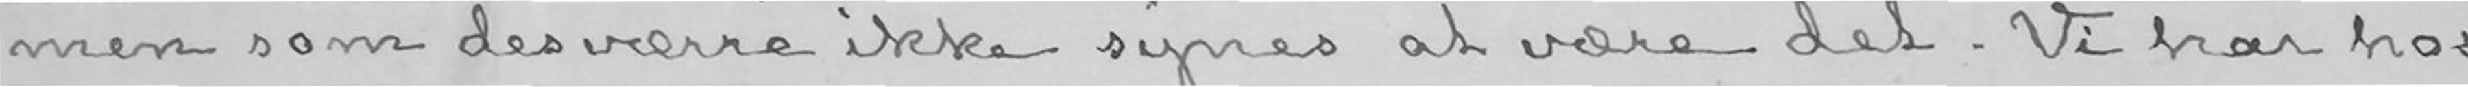men som desværre ikke synes at være det. Vi har hos
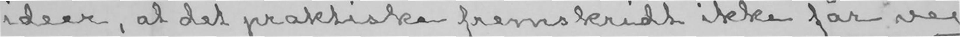ideer, at det praktiske fremskridt ikke får vej
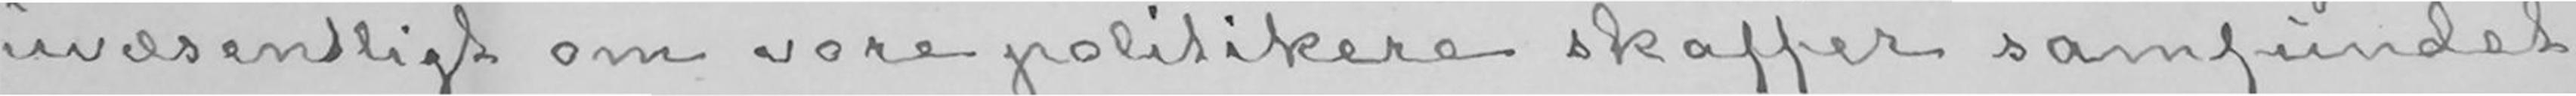uvæsentligt om vore politikere skaffer samfundet
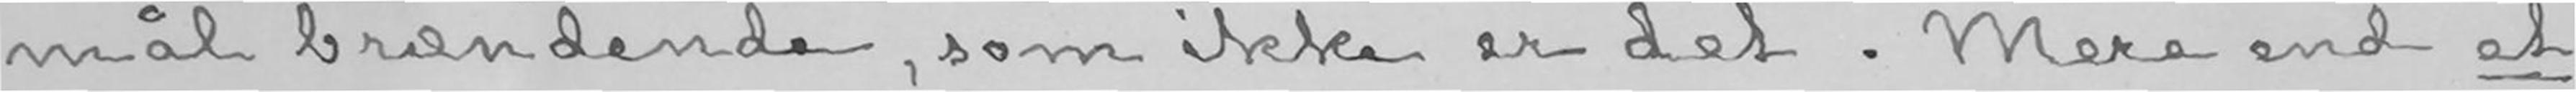mål brændende, som ikke er det. Mere end et
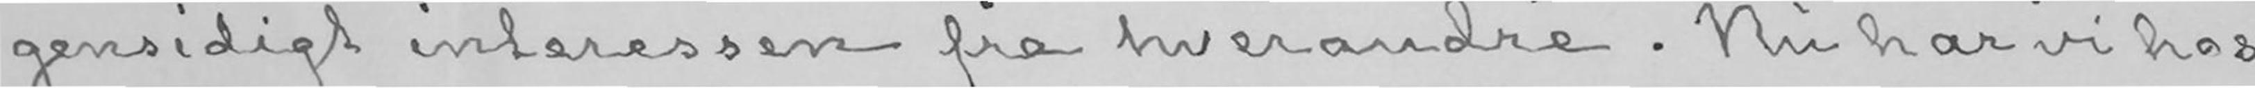gensidigt interessen fra hverandre. Nu har vi hos
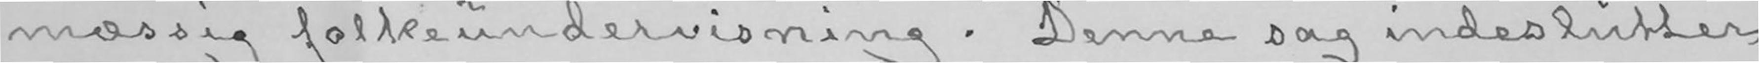mæssig folkeundervisning. Denne sag indeslutter
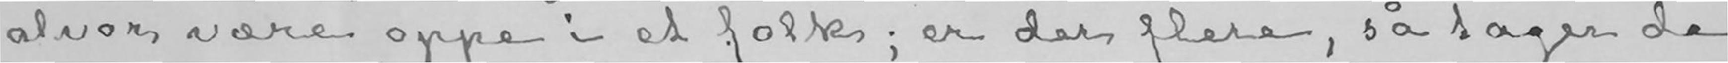alvor være oppe i et folk; er der flere, så tager de
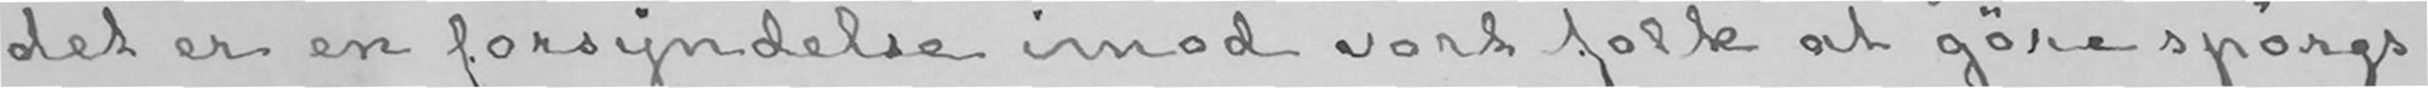det er en forsyndelse imod vort folk at gøre spørgs-
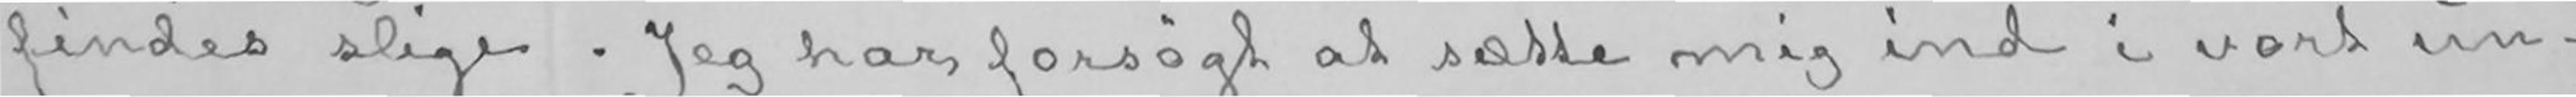findes slige. Jeg har forsøgt at sætte mig ind i vort un-
